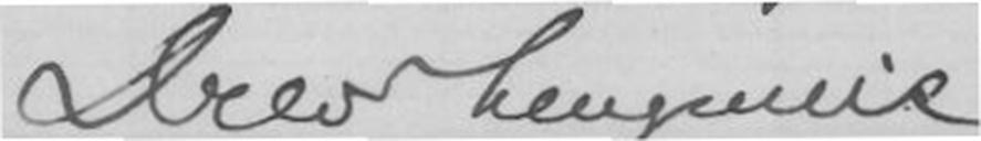Deres hengivne
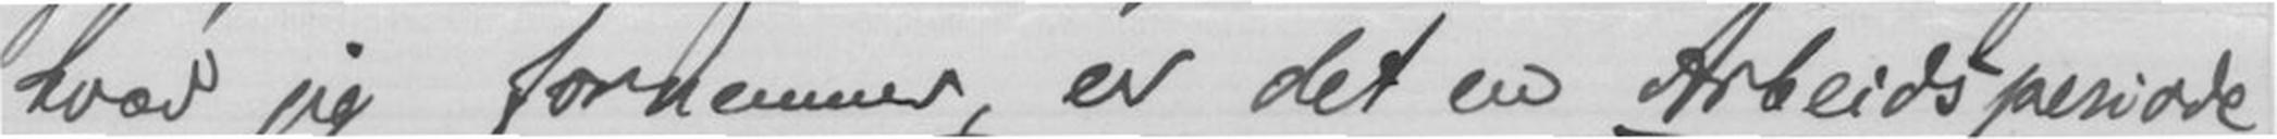hvad jeg fornemmer, er det en Arbeidsperiode,
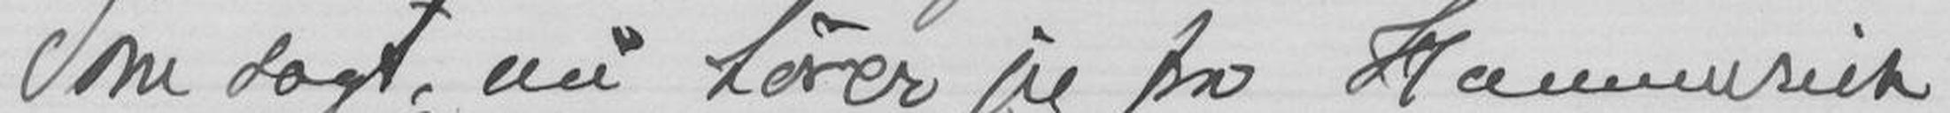Som sagt, nu hører jeg fra Hammerick
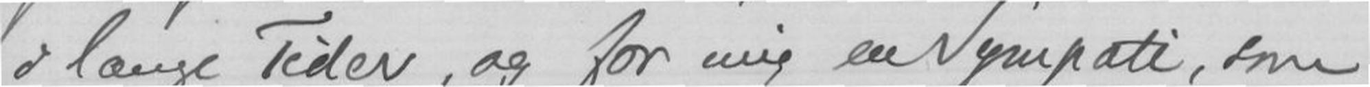i lange Tider, og for mig en Sympati, som
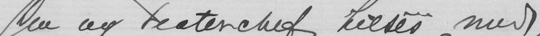De og Teaterchef hilses med
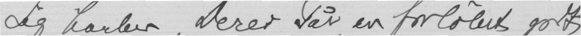Jeg haaber, Deres Tur er forløbet godt,
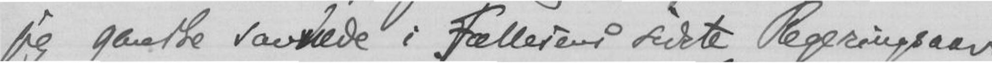jeg ganske savnede i Fælles end sidste Regeringsaar.
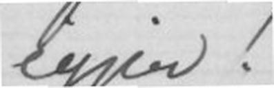igjen!
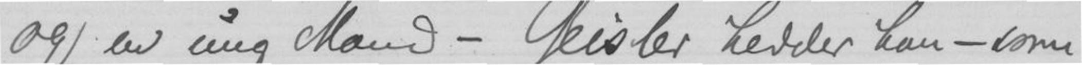og en ung Mand - Geisler hevder han -
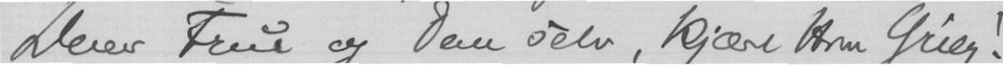Deres Frue og Dem selv, kjære Herr Grieg!
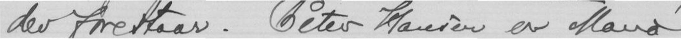der forestaar. Peter Hansen er Mand
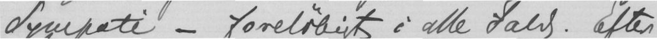Sympati - foreløbigt i alle Falds. Efter
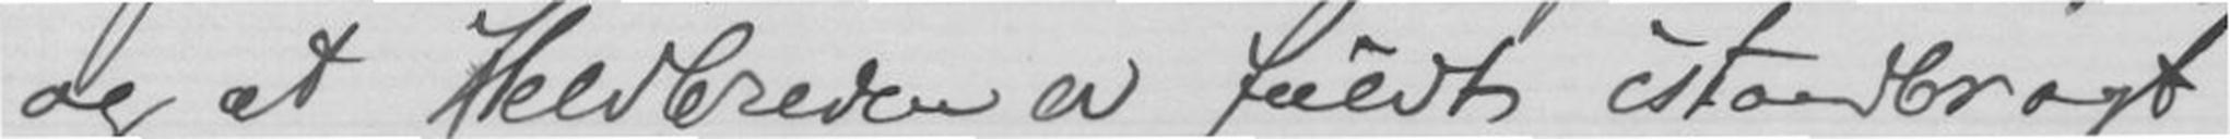og at Heldbreden er fuldt istandbragt
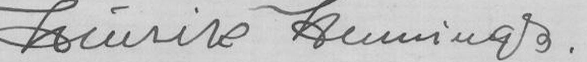Henrik Hennings.
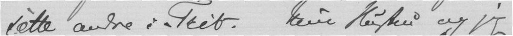sætte andre i Tri.. Min Hustru og jeg
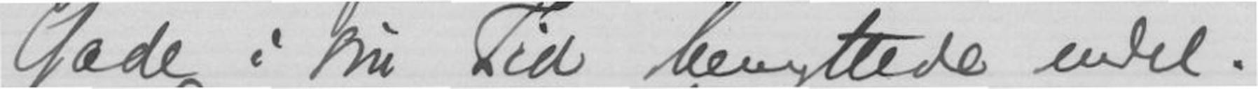Gade i nu Tid benyttede endel.
